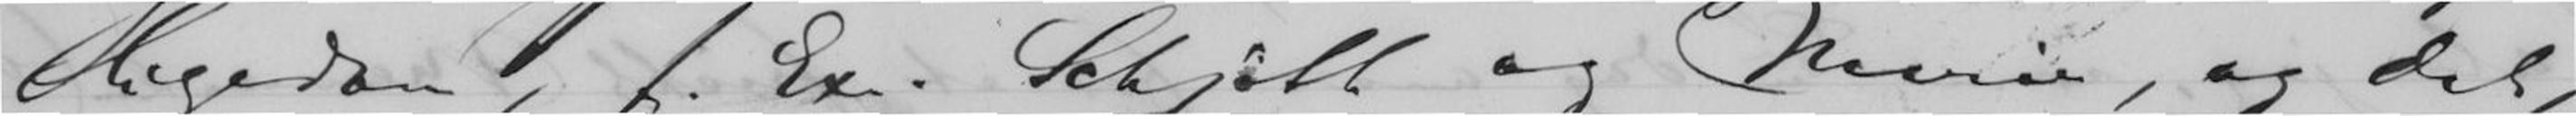ligedan, f. Ex. Schjøtt og Marie, og det
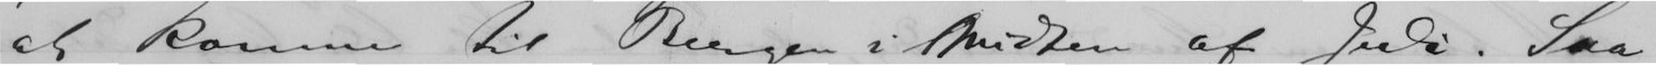at komme til Bergen i Midten af Juli. Saa
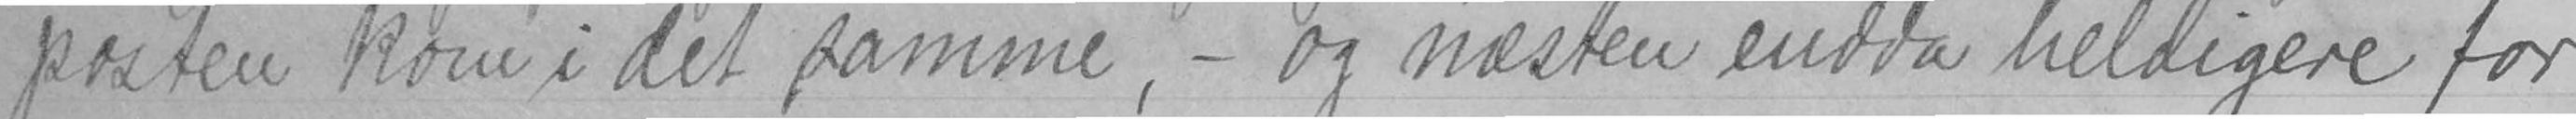posten kom i det samme, - og næsten endda heldigere for
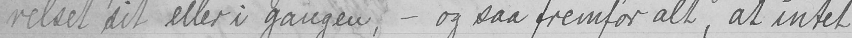relset sit eller i gangen; - og saa fremfor alt, at intet
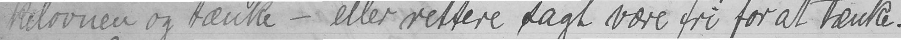kelovnen og tænke - eller rettere sagt være fri for at tænke.
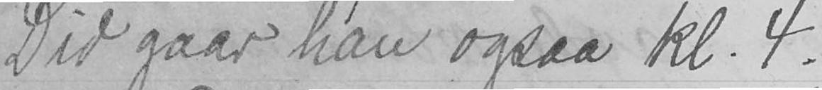Did gaar han ogsaa kl. 4.
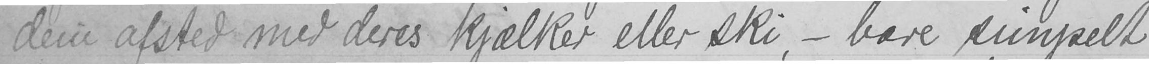dem afsted med deres kjælker eller ski, - bare simpelt
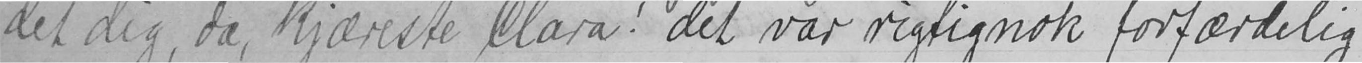det dig, da, kjæreste Clara! det var rigtignok forfærdelig
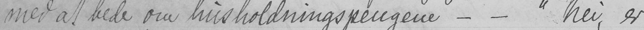med at bede om husholdningspengene - - "Nei, er
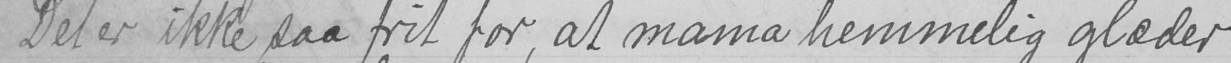Det er ikke saa frit for, at mama hemmelig glæder
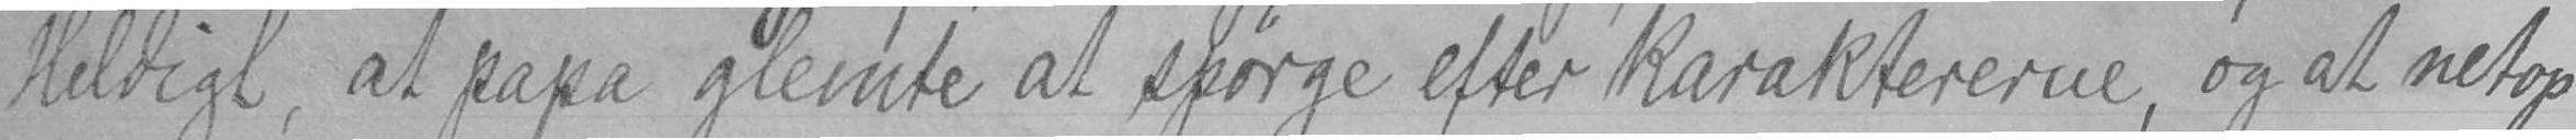Heldigt, at papa glemte at spørge efter karaktererne, og at netop
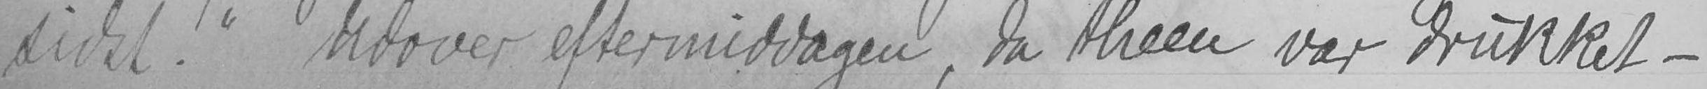sidst." Udover eftermiddagen, da theen var drukket -
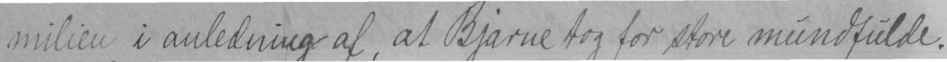milien i anledning af, at Bjarne tog for store mundfulde.
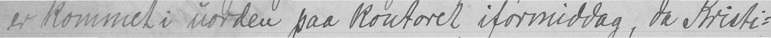er kommet i uorden paa kontoret iformiddag, da Ktristi-
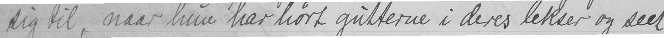sig til, naar hun har hørt gutterne i deres lekser og seet
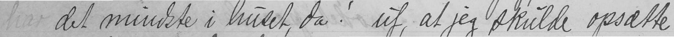har det mindste i huset, da! uf, at jeg skulde opsætte
